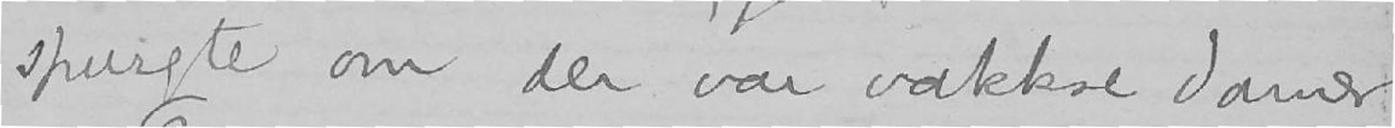spurgte om der var vakkre damer
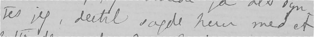tes jeg, dertil sagde hun med et
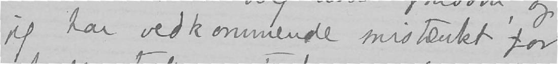jeg har vedkommende mistænkt for
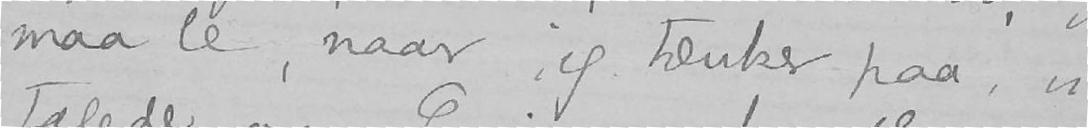maa le, naar jeg tænker paa, vi
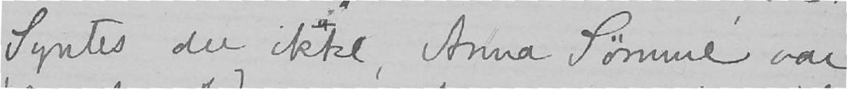Syntes du ikke, Anna Sømme var
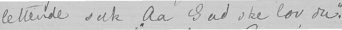lettende suk «Aa Gud ske lov du.»
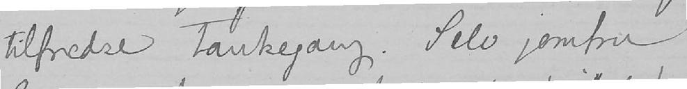tilfredse tankegang. Selv jomfru
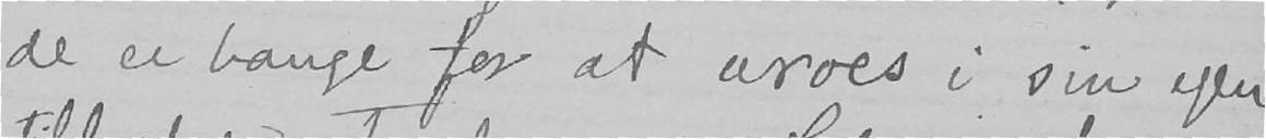de er bange for at uroes i sin egen
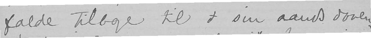falde tilbage til d sin aands doven-
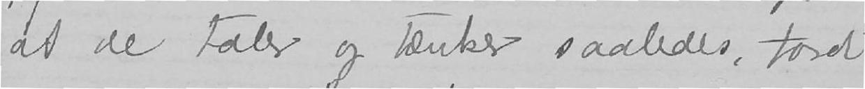at de taler og tænker saaledes, fordi
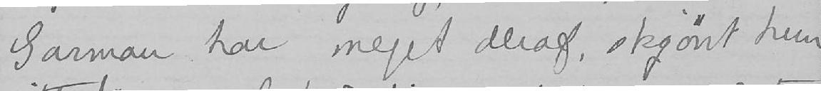Garman har meget deraf, skjønt hun
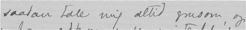saadan tale mig altid grusom, og
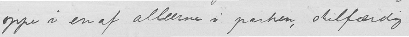oppe i en af alleerne i parken, stilfærdig
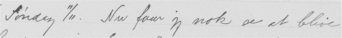Søndag 11/11. Nu faar jeg nok se at blive
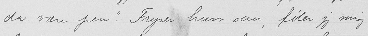da være pen". Fryser hun saa, føler jeg mig
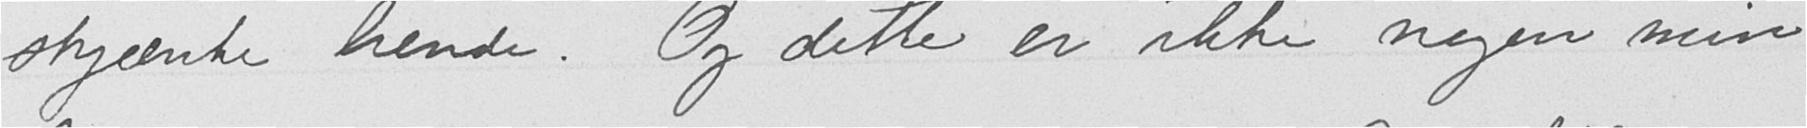skjænke hende. Og dette er ikke nogen min
